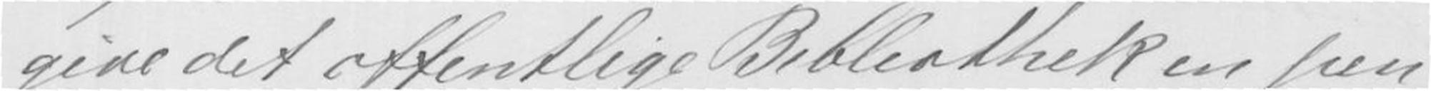give det offentlige Bibleothek en saa
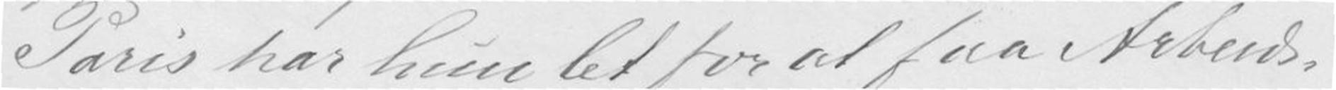Paris har hun let for at faa Arbeids-
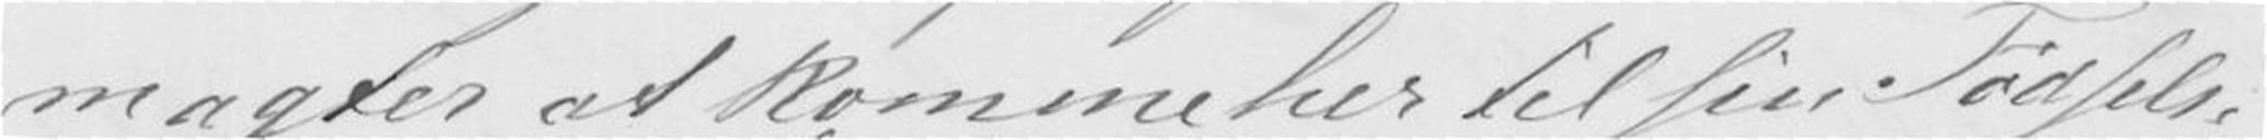magter at komme her til sin fødsels-
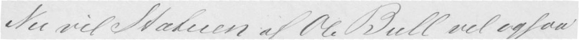Nu vil Statuen af Ole Bull vel ogsaa
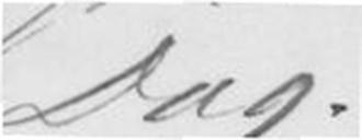Dag.
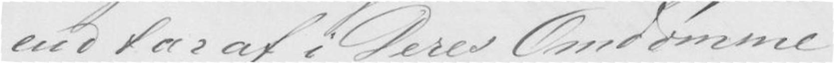end tar af i Deres Omdømme.
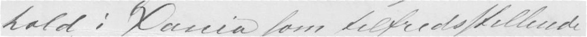hold i Xania som tilfredstillende
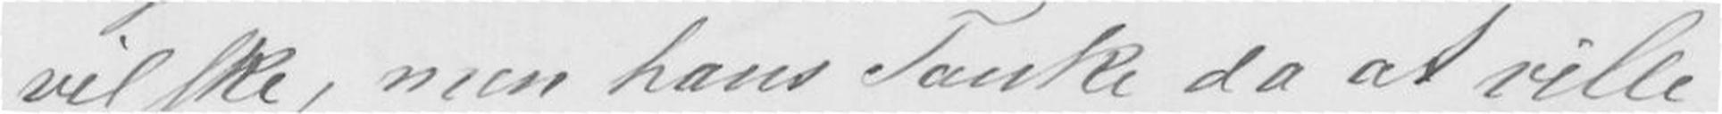vil ske, men hans Tanke da at ville
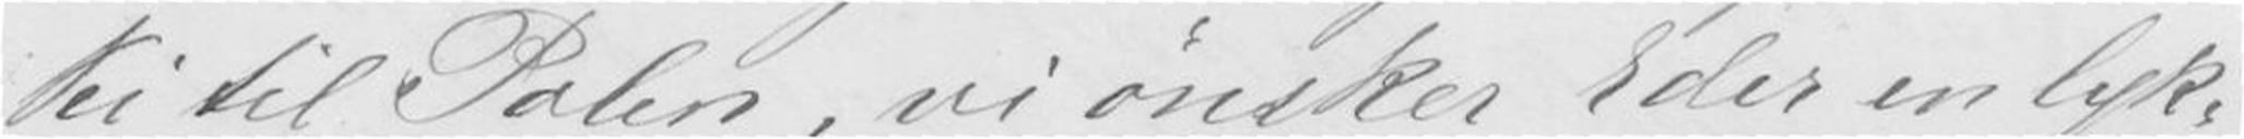vei til Polen, vi ønsker Eder en luk-
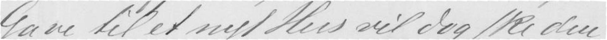Gave til et nyt Hus vil dog ske den
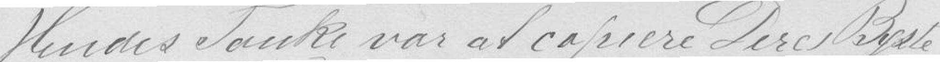Hendes Tanke var at caplere Deres byste
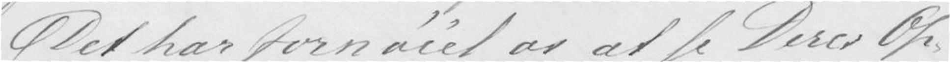Det har fornøiet av at se Deres Op-
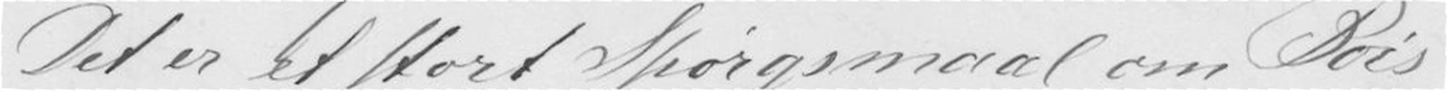Det er et stort Spørgsmaal om Børs
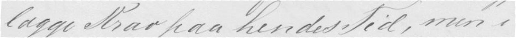lægge Krav paa hendes Tid, men i
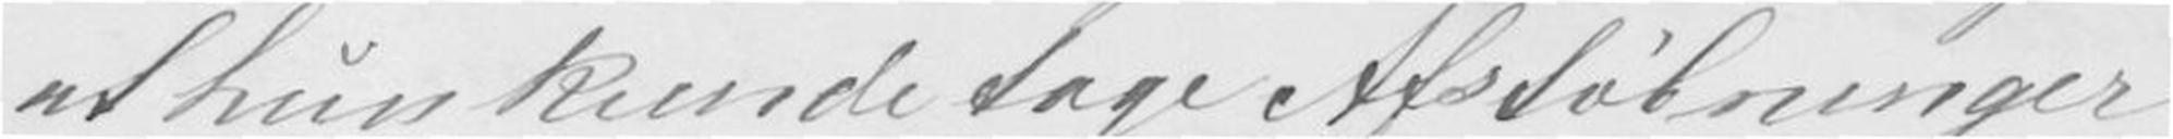at hun kunde tage Afstøbninger
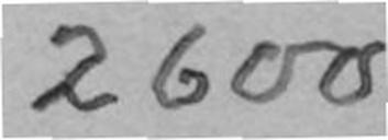2600
